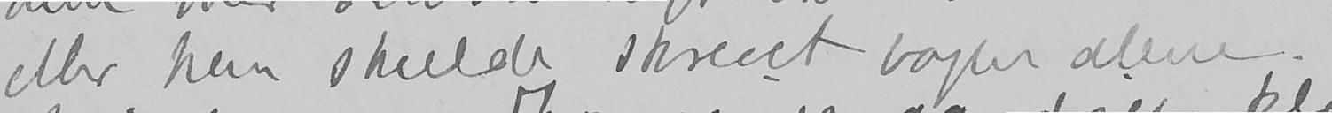eller han skulde skrevet bogen alene.
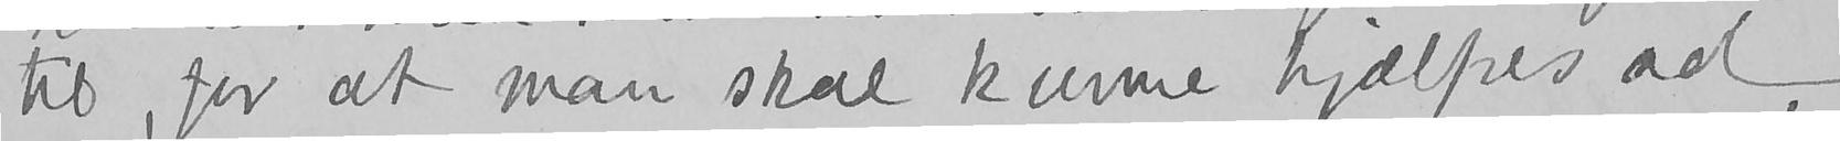til, for at man skal kunne hjælpes ad,
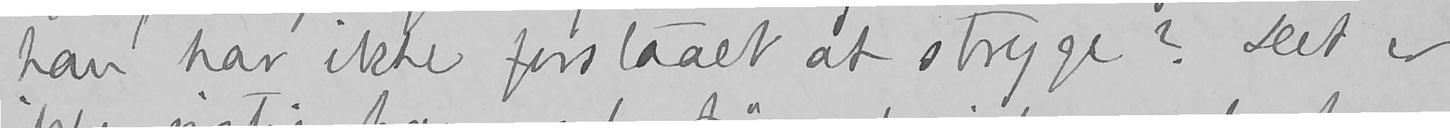han har ikke forstaaet at stryge? Det er
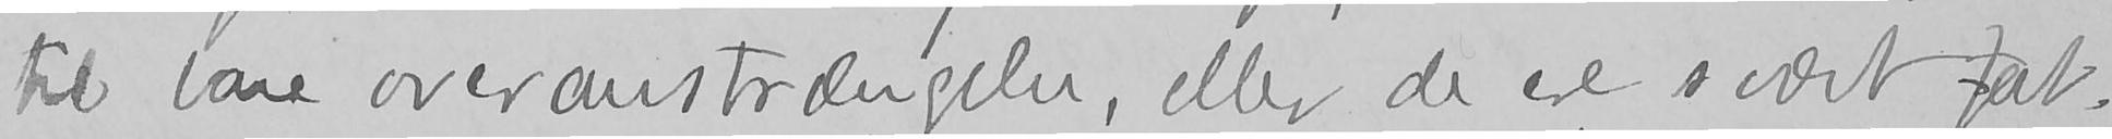til bare overanstrængelse, eller de ere svært fat-
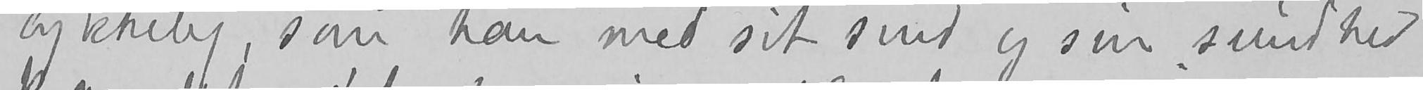lykkelig, som han med sit sind og sin sundhed
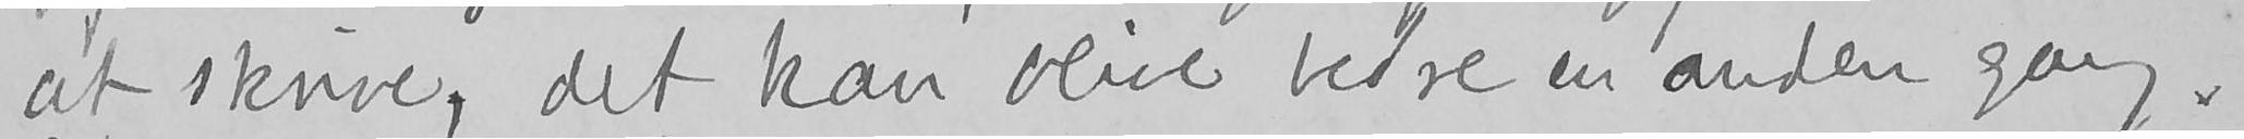at skrive, det kan blive bedre en anden gang.
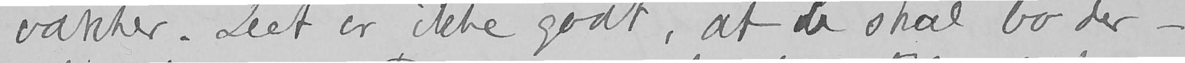vakker. Det er ikke godt, at de skal bo der
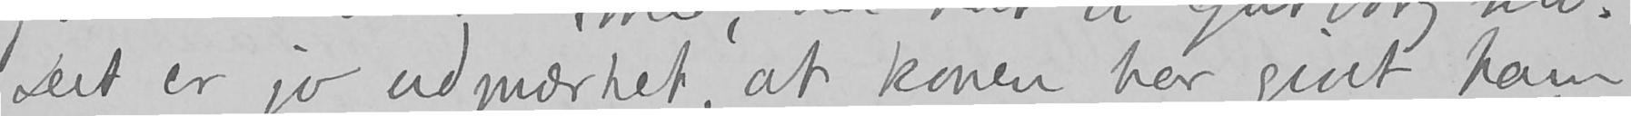Det er jo udmærket, at konen har givet ham
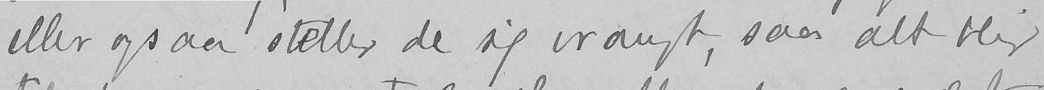eller ogsaa steller de sig vrangt, saa alt blir
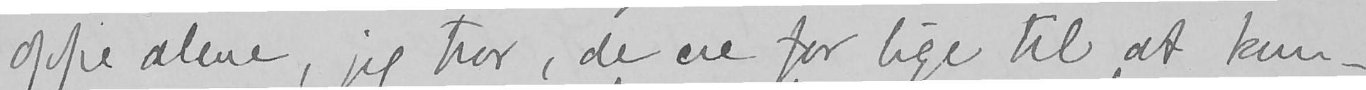oppe alene, jeg tror, de ere for lige til at kun-
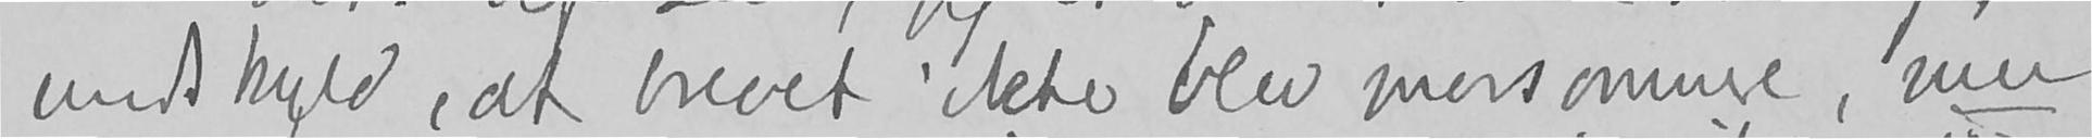undskyld, at brevet ikke blev morsomme, men
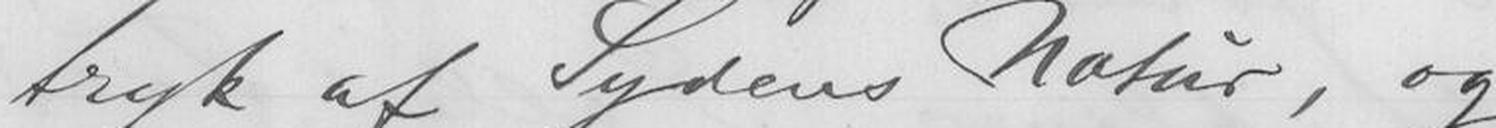tryk af Sydens Natur, og
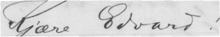Kjære Edvard!
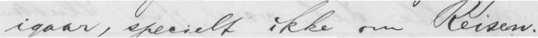igaar, specielt ikke om Reisen.
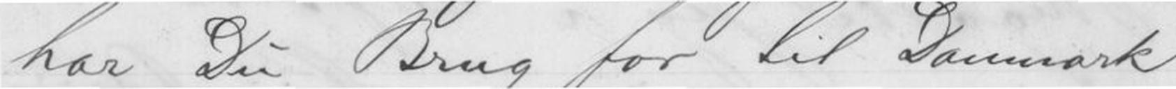har Du Brug for til Danmark
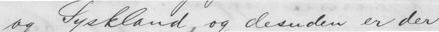og Tyskland, og desuden er der
